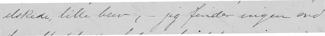elskede, lille brev, - jeg finder ingen ord
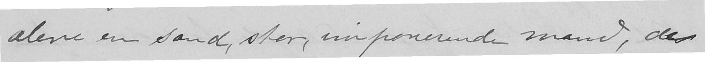alene en sand, stor, imponerende mand, de
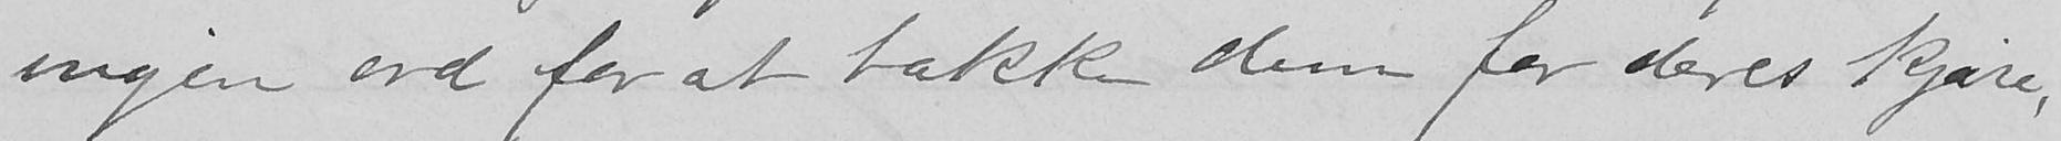ingen ord for at takke dem for deres kjære,
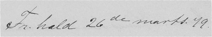Fr. hald 26de marts. 79.
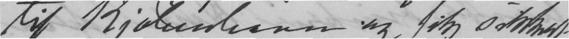til Kjøbenhavn og, fik sikkert
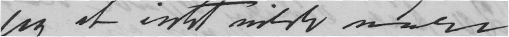jeg at intet vilde være
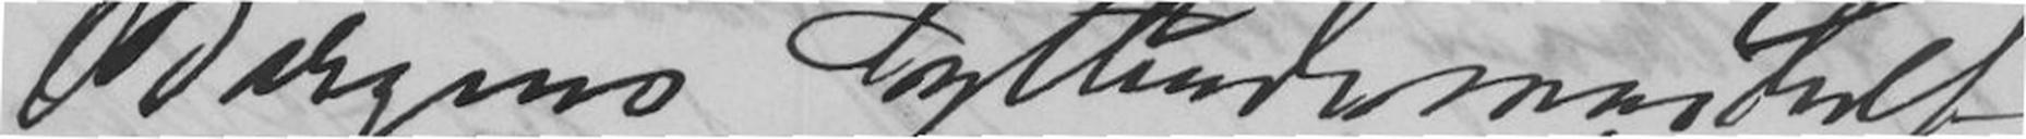Bergens Syttendemaihelt
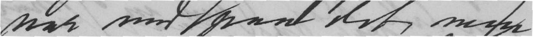var med paa det, men
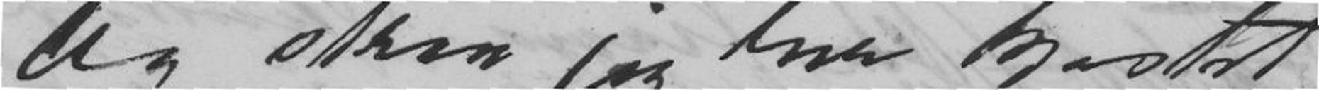Og strax jeg her kastet
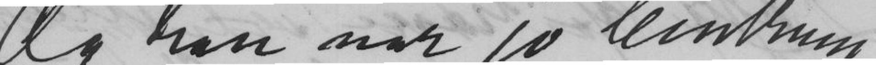Og han var jo Centrum
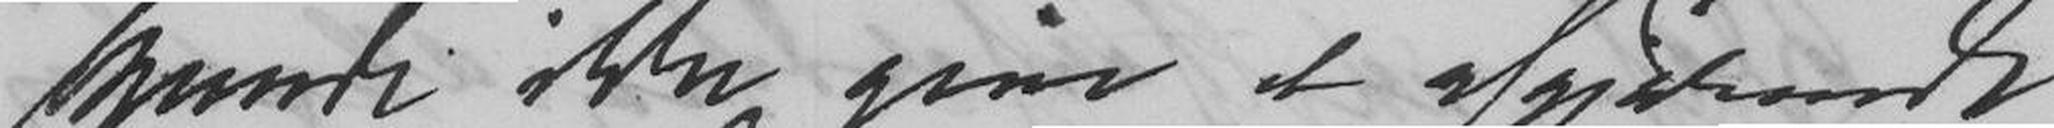kunde ikke give et afgjørende
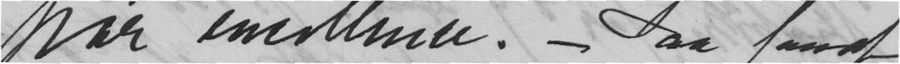par excellence.- Saa fandt
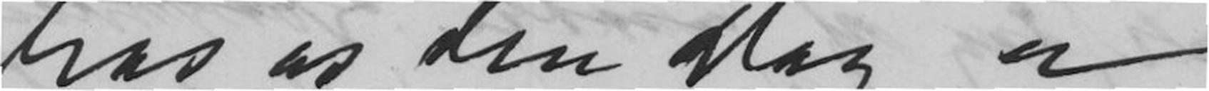hos os den Dag og
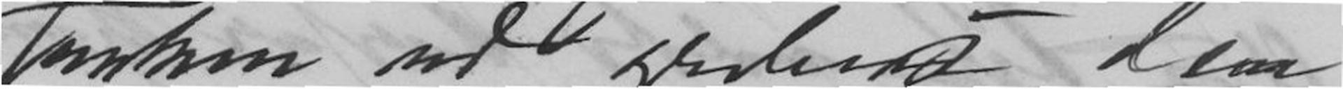Tanken ud grebes den
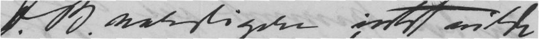O. B. naturligere intet vilde
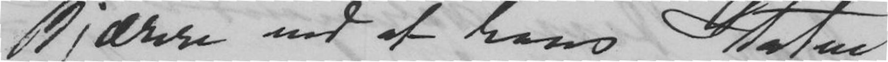kjærere end at hans statue
